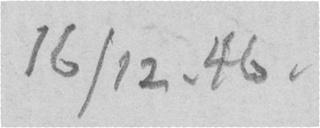16/12. 46.
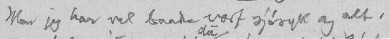Men jeg har vel baade vært sjøsyk og alt,
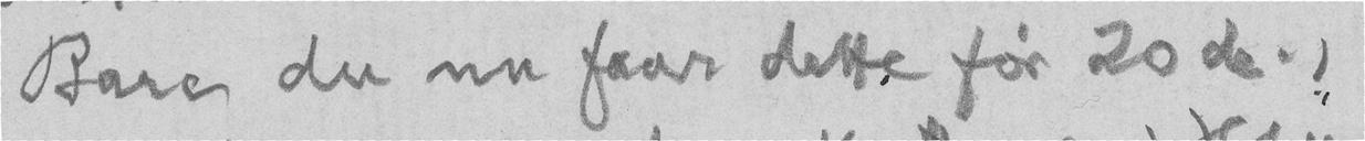Bare du nu faar dette før 20de.,
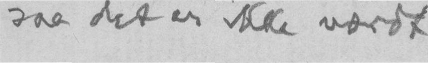saa det er ikke værdt
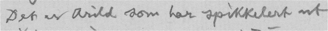Det er Arild som har spikkelert ut
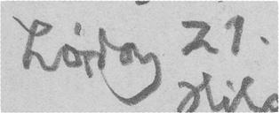Lørdag 21.
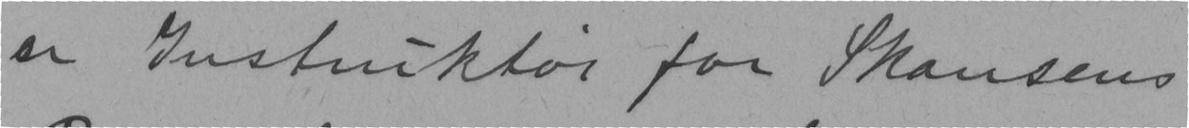er Instruktør for Skansens
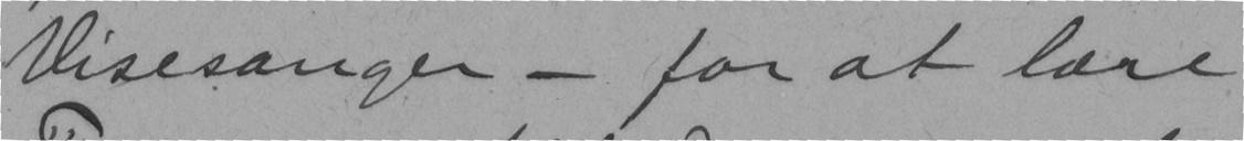Visesanger - for at lære
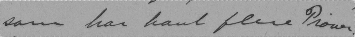som har havt flere Prøver.
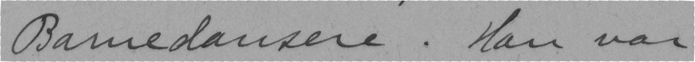Barnedansere. Han var
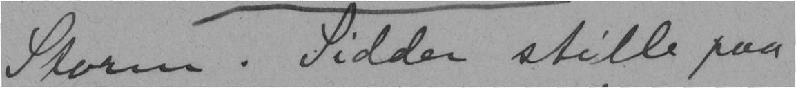Storm. Sidder stille paa
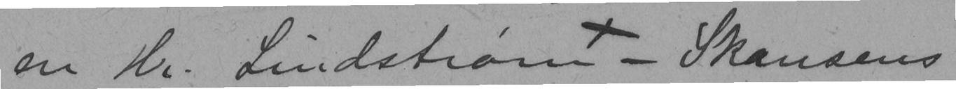en Hr. Lindstrøm - Skansens
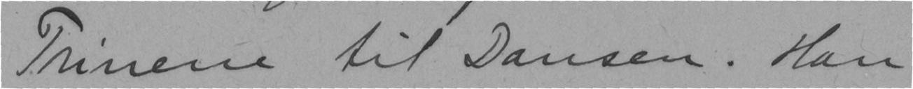Trinene til Dansen. Han
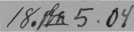18. 5.04
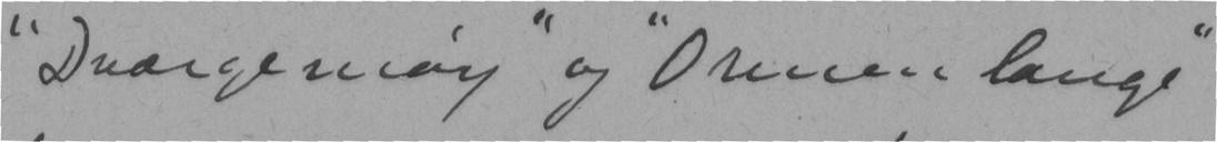"Dværgemøy" og "Ormen lange"
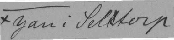x Jan i Seltorp
